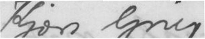Kjære Grieg!
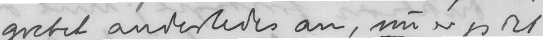grebet anderledes an, nu er jeg det
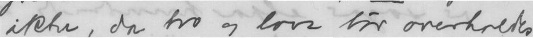ikke, da tro og love bør overholdes,
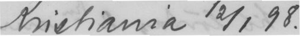Kristiania 12/1 98
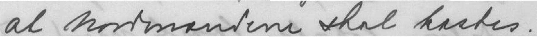at Nordmændene skal kastes.
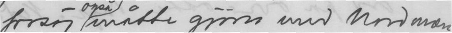forsøg måtte gjøres med Nordmæn-
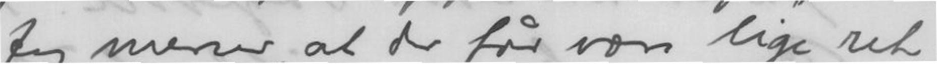Jeg mener, at der får være lige ret
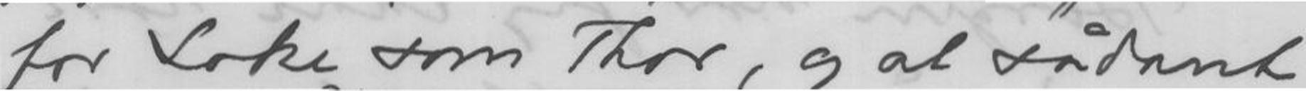for Loke som Thor, og at sådant
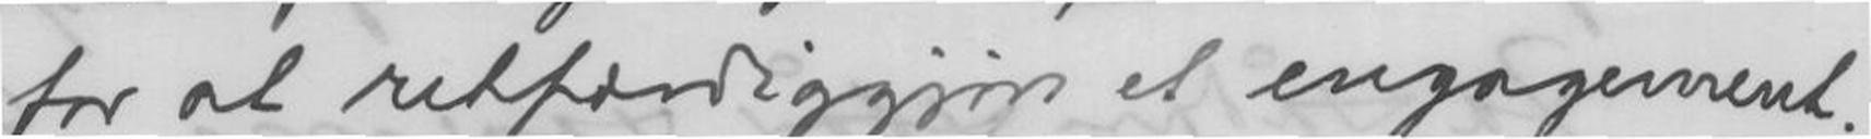for at retfærdiggjøre et engagement.
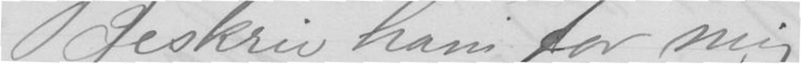Beskriv ham for mig
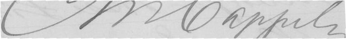Chr. Cappelen
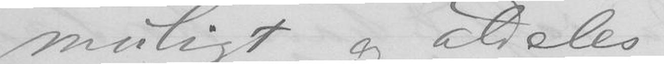muligt og aldeles
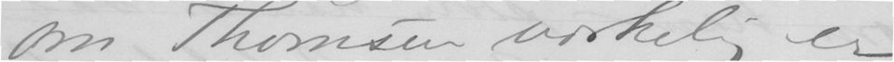om Thomsen virkelig er
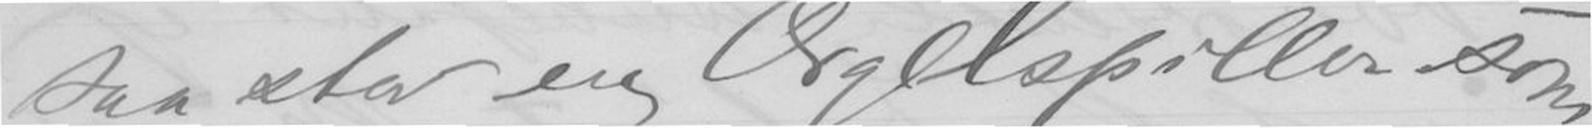saa stor ung Orgelspiller som
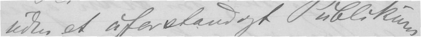uden et uforstandigt Publikums
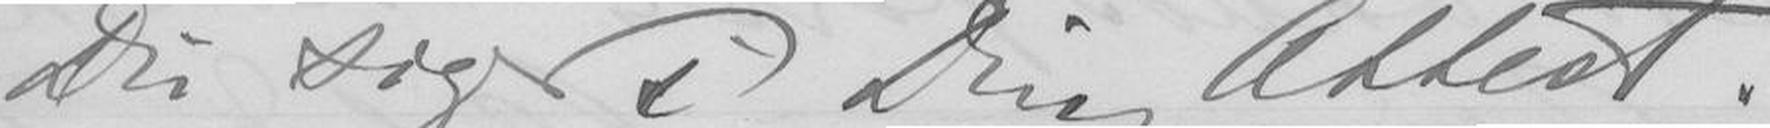Du siger i Din Attest.
